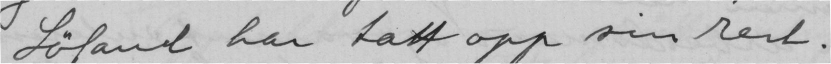Løland har tatt opp sin rest.
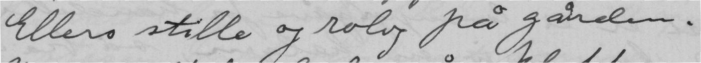Ellers stille og rolig på gården.
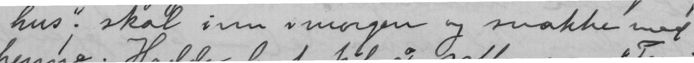hus"; skal inn imorgen og snakke med
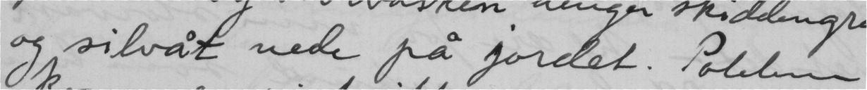og silvåt nede på jordet. Potetene
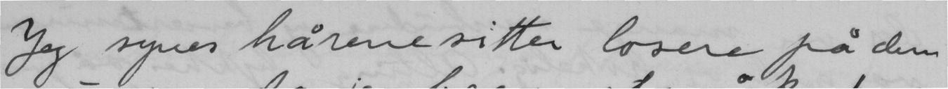Jeg synes hårene sitter løsere på dem
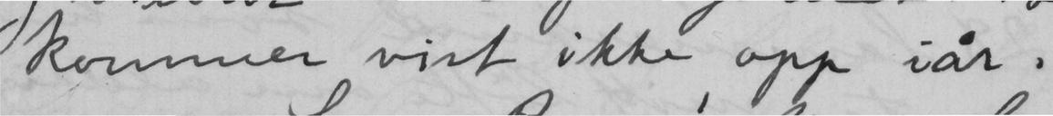kommer vist ikke opp iår.
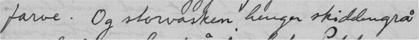farve. Og storvasken henger skiddengrå
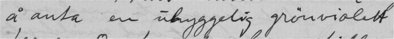å anta en uhyggelig grønviolett
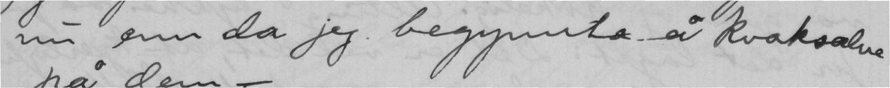nu enn da jeg begynnte å kvaksalve
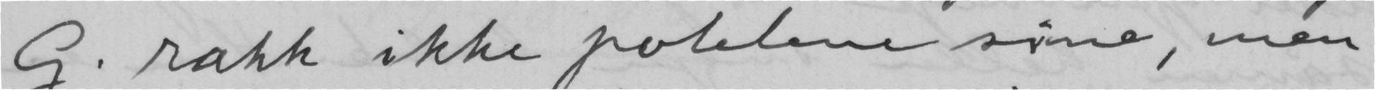G. rakk ikke potelene sine, men
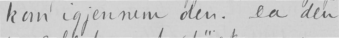kom igjennem den. Da den
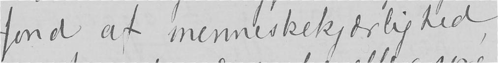fond af menneskekjærlighed,
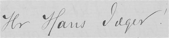Hr Hans Jæger!
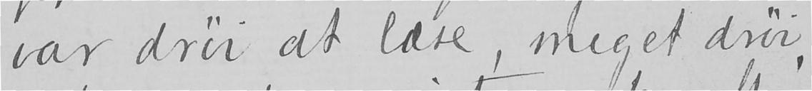var drøi at læse, meget drøi,
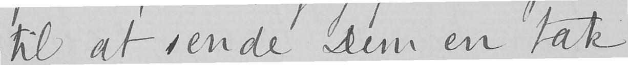til at sende Dem en tak
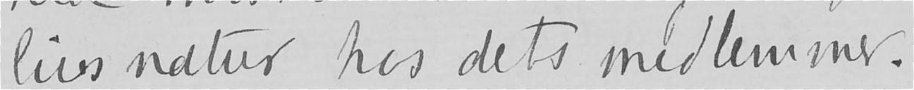livs natur hos dets medlemmer.
